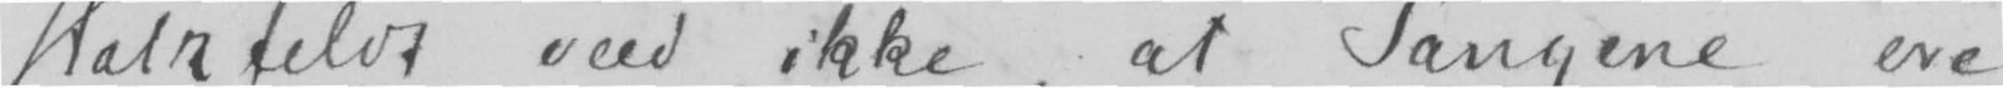Hatzfeldt ved ikke, at Sangene ere
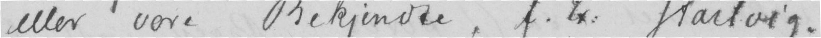eller vore Bekjendte, f.Ex: Hartvig-
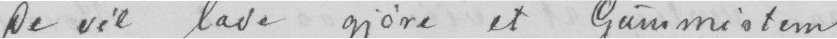De vil lade gjøre et Gummistem
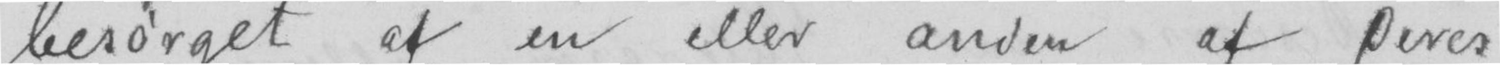besørget af en eller anden af Deres
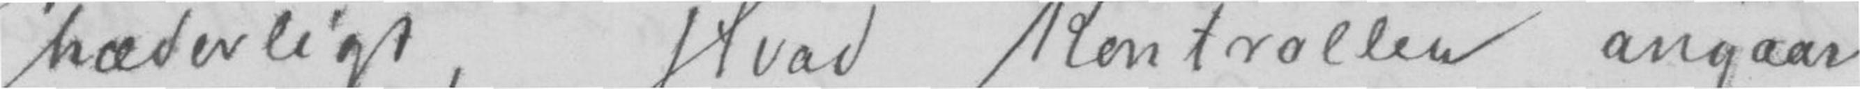hæderligt. Hvad Kontrollen angaar
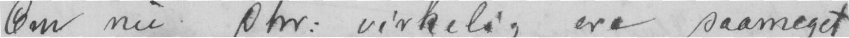Om nu Dhr virkelig ere saameget
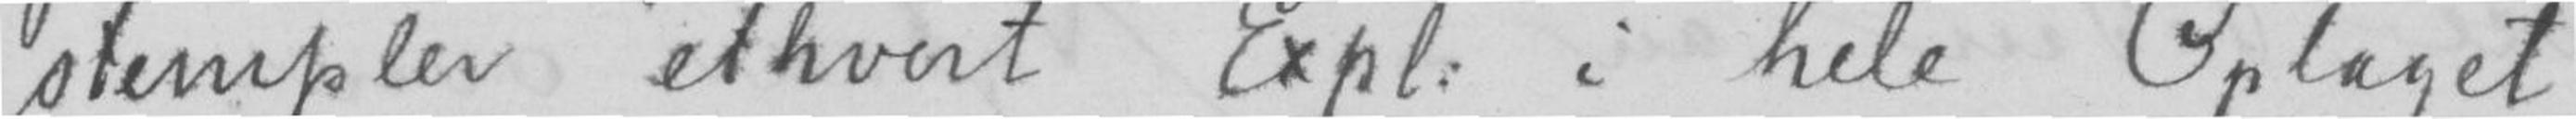stempler ethvert Expl: i hele Oplaget
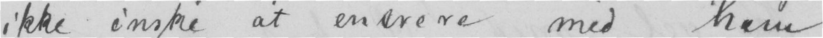ikke inske at endrere med ham
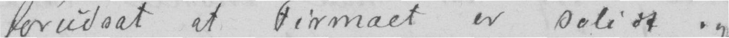forudsat at Firmaet er solidt og
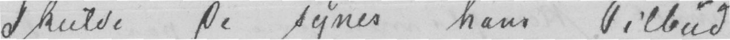Skulde De synes hans Tilbud
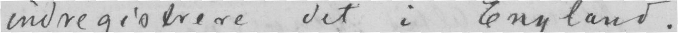indregistrer det i England.
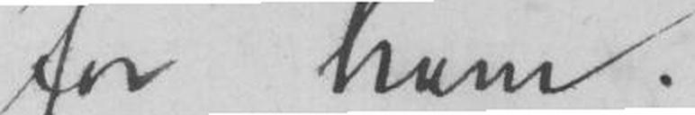for ham.
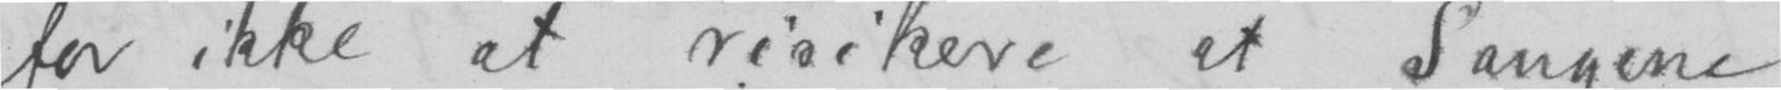for ikke at risikere at Sangene
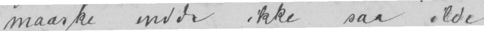maaske endnu ikke saa ilde
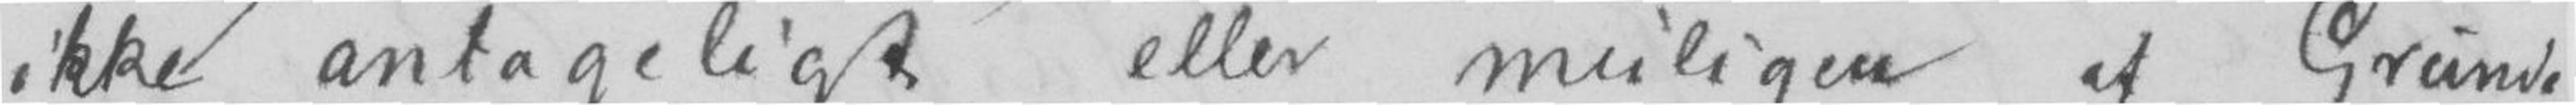ikke antageligt eller muligen af Grunde
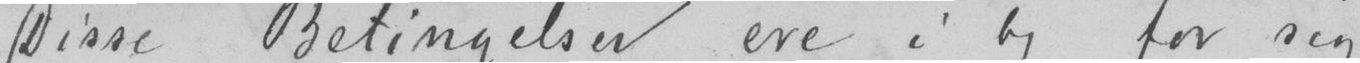Disse Betingelser ere i og for sig
